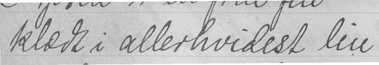klædt i allerhvidest lin
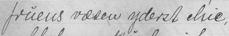fruens væsen yderst chic,
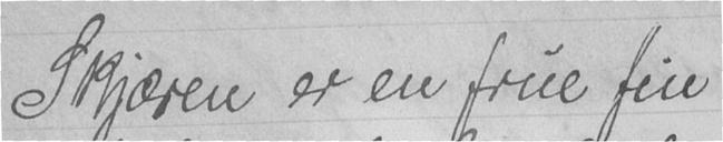Skjæren er en frue fin
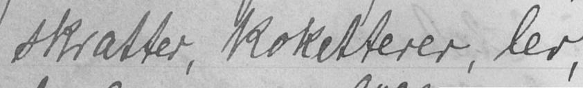skratter, koketterer, ler,
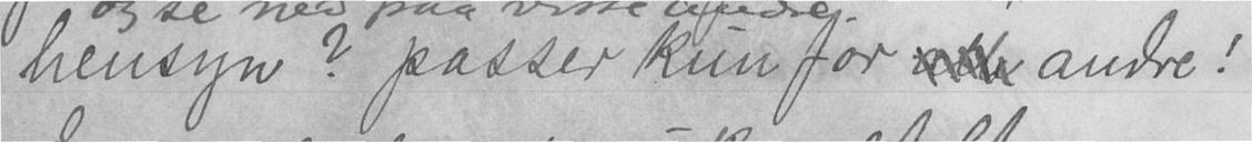hensyn? passer kun for alle andre!
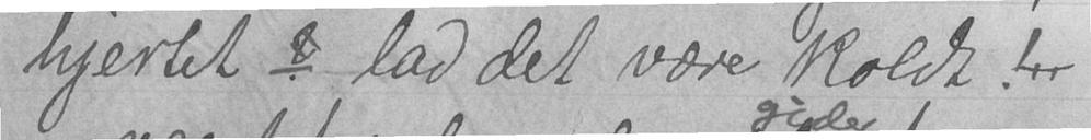hjertet? - lad det være koldt. !..
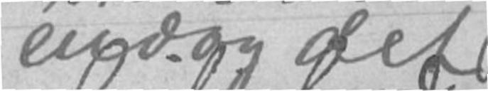endog det
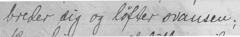breder sig og løfter svansen;
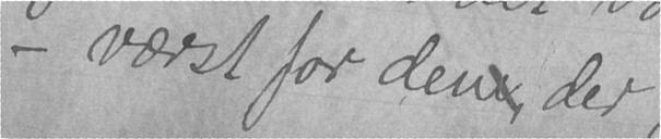- værst for denm, der
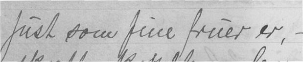Just som fine fruer er, -
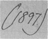(1897)
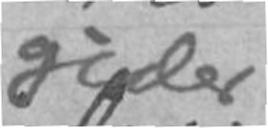gider
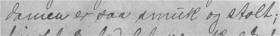damen er saa smuk og stolt;
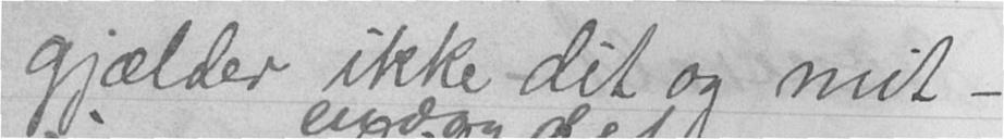gjælder ikke dit og mit -
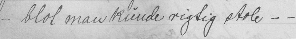- blot man kunde rigtig stole - -
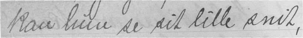kan hun se sit lille snit,
